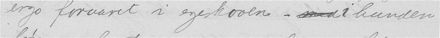ergo foraaret i egeskoven - med i lunden
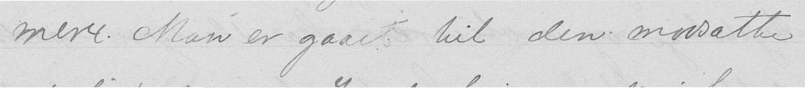mere. Man er gaaet til den modsatte
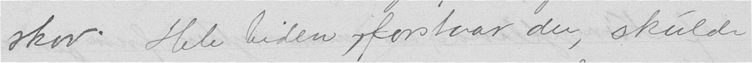skov. Hele tiden, forstaar du, skulde
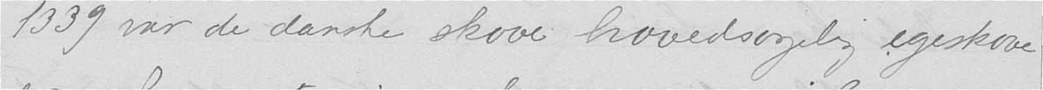1339 var de danske skove hovedsagelig egeskove
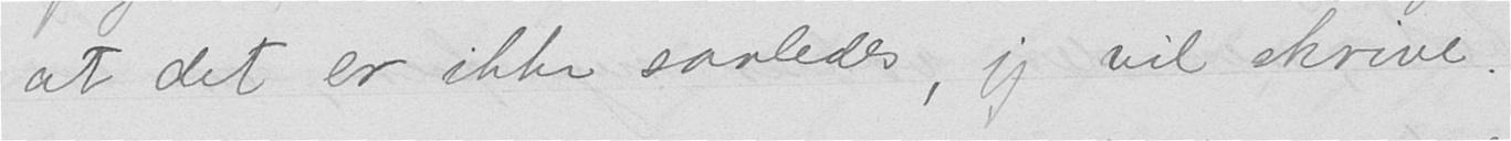at det er ikke saaledes,  jeg vil skrive.
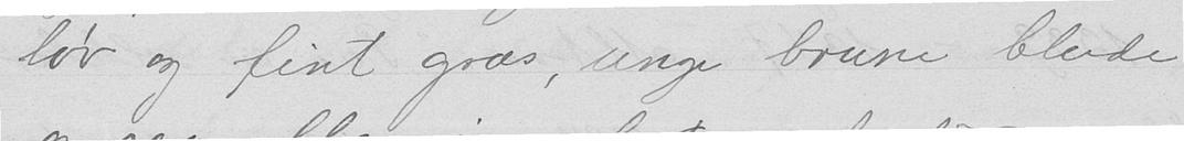løv og fint græs, unge brune blade
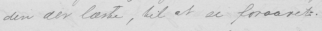den der læste, til at se foraaret.
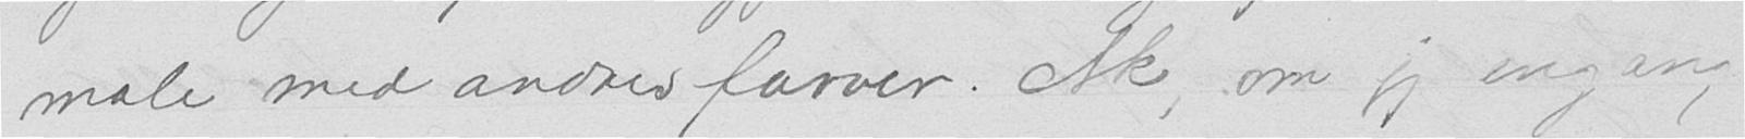male med andres farver. Ak, om jeg engang
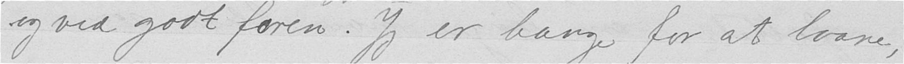og ved godt faren. Jeg er bange for at laane,
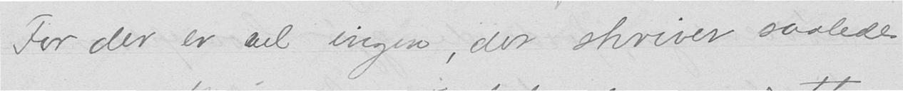For der er vel ingen, der skriver saaledes
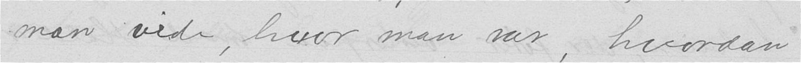man vide, hvor man var, hvordan
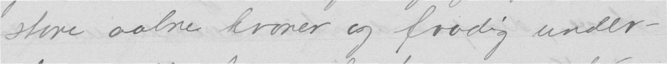store aabne kroner og frodig under-
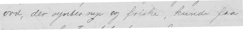ord, der syntes nye og friske, kunde faa
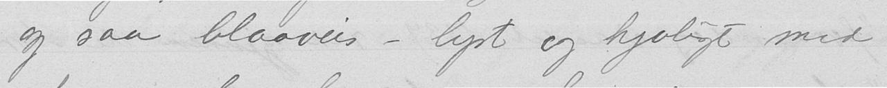og saa blaaveis - lyst og kjøligt med
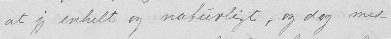at jeg enkelt og naturligt, og dog med
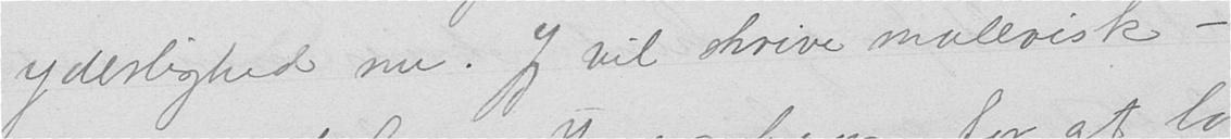yderlighed nu. Jeg vil skrive malerisk -
